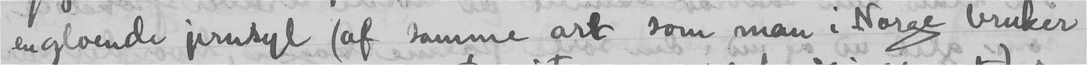en gloende jernsyl (af samme art som man i Norge bruker
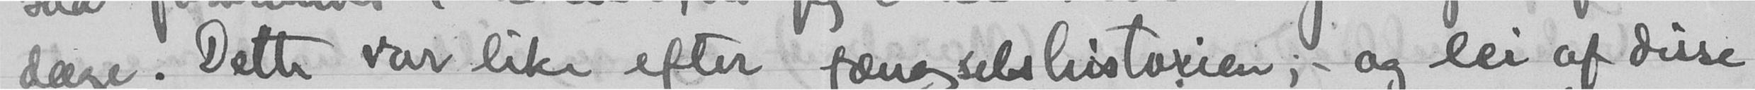dage. Dette var like efter fængselshistorien; - og lei af dise
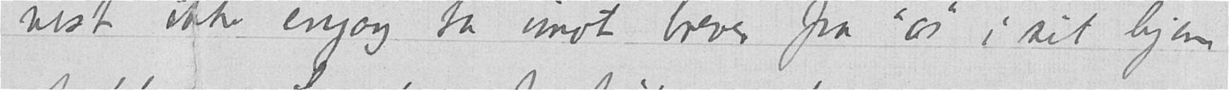vist ikke engang ta imot brever fra "os" i sit hjem
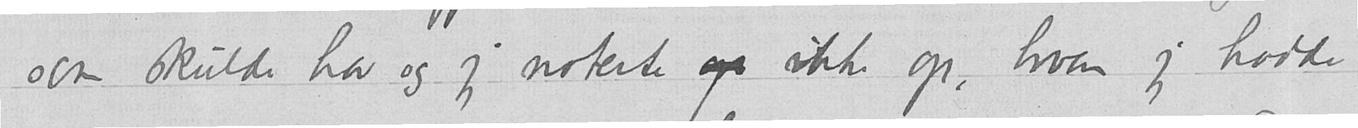som skulde ha og jeg noterte op ikke op, hvem jeg hadde
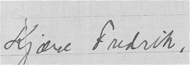Kjære Fredrik,
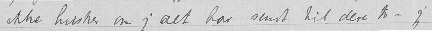ikke husker om jeg alt har sendt til dere to – jeg
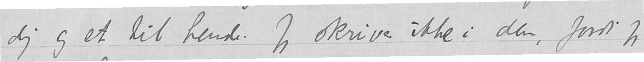dig og et til hende. Jeg skriver ikke i dem, fordi jeg
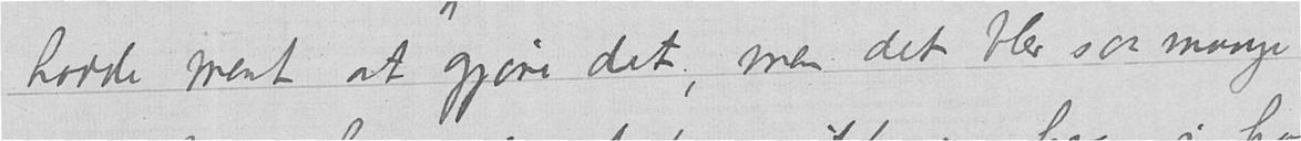hadde ment at gjøre det, men det blev saa mange
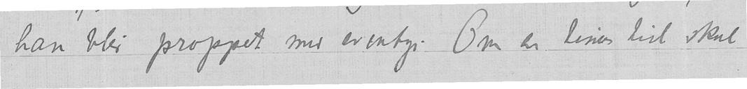han blir proppet med eventyr. Om en times tid skal
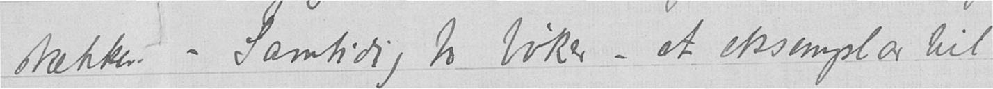stakkar. – Samtidig to bøker – et eksemplar til
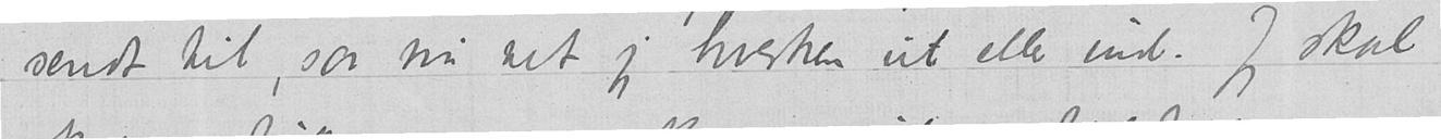sendt til, saa nu vet jeg hverken ut eller ind. Jeg skal
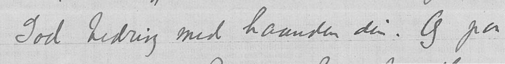God bedring med haanden din. Og paa
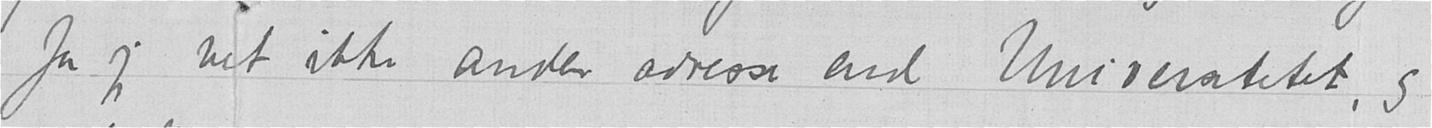for jeg vet ikke anden adresse end Universitetet, og
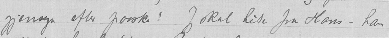gjensyn efter paaske! Jeg skal hilse fra Hans – han
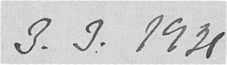3.3.1931
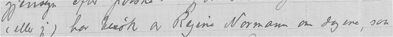(eller jeg) har besøk av Regine Normann om dagene, saa
